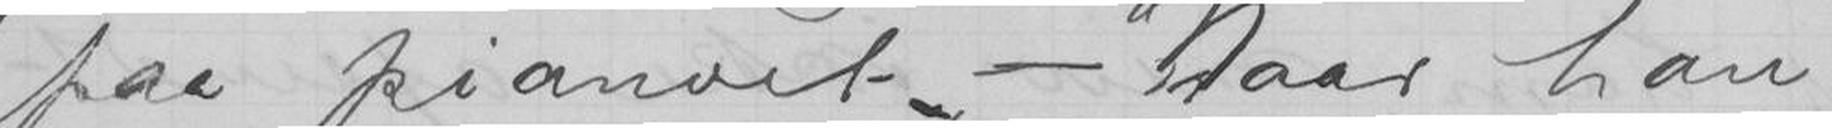paa pianoet,- Naar han
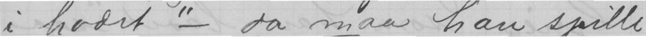i hodet- da maa han spille,
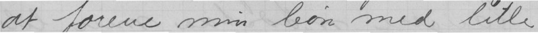at forene min bøn med lille
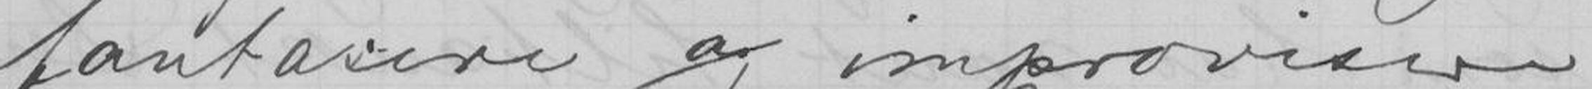fantasere og improvisere
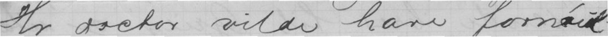Hr Doctor, vilde have fornøiel-
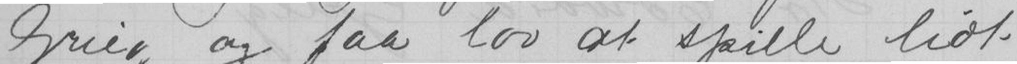Grieg og faa lov at spille lidt
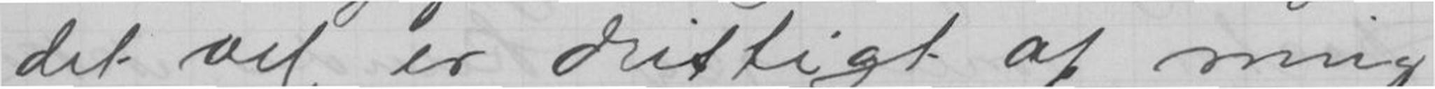det vel er dristigt af mig
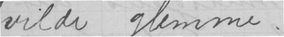vilde glemme.
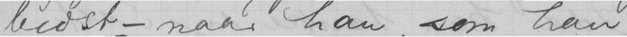bedst,- naar han som han
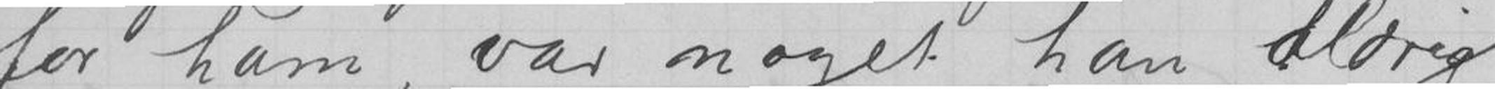for ham, var noget han aldrig
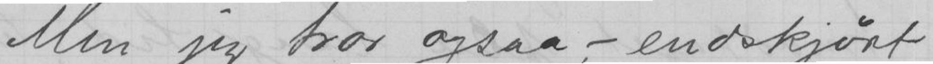Men jeg tror ogsaa,- endskjønt
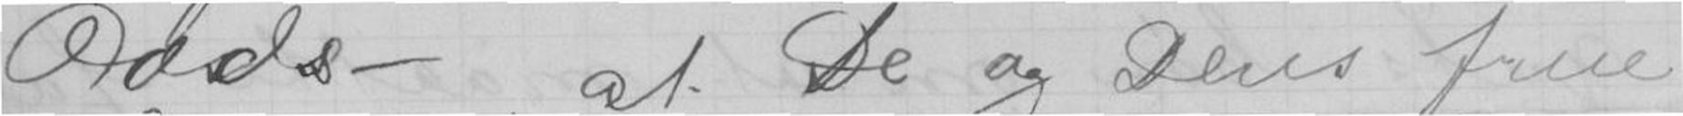Odds- at De og Deres frue
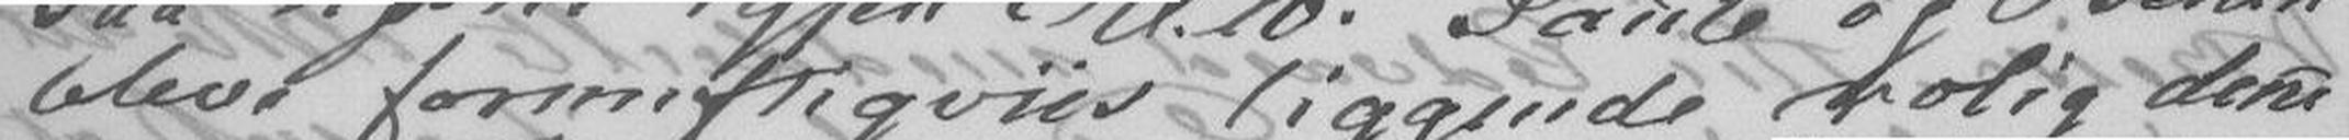bleve fornuftigviis liggende rolig de`ne
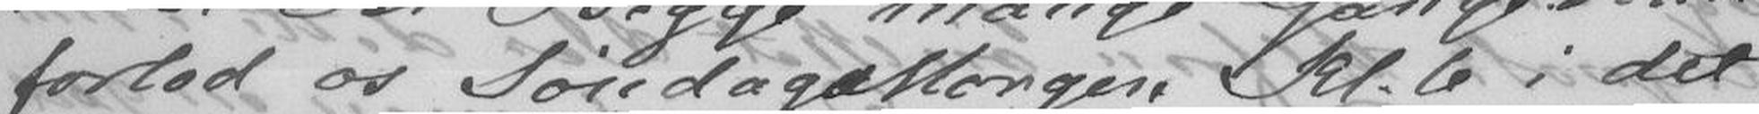forlod os Søndags Morgen Kl.6 i det
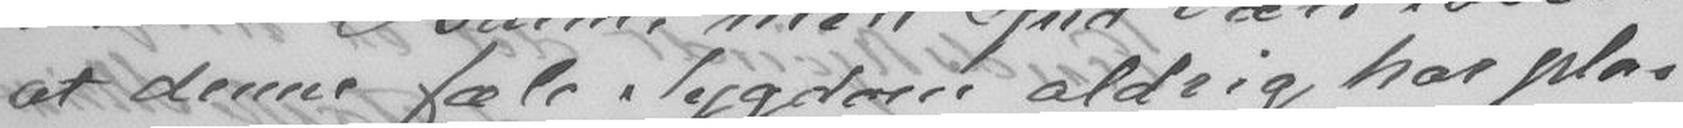at denne fæle Sygdom aldrig har pla-
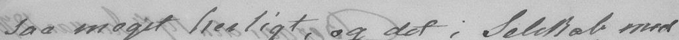saa meget herligt, og det i Selskab med
